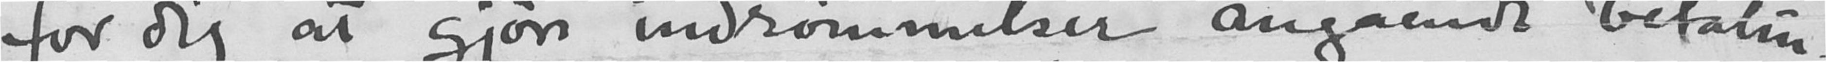for dig at gjøre indrømmelser angående betalin-
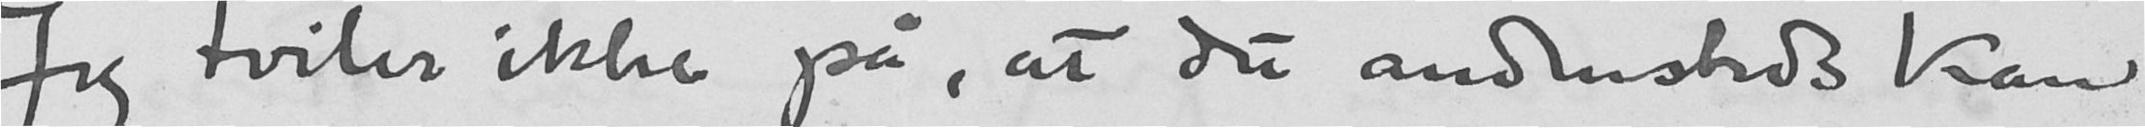Jeg tviler ikke på, at du andensteds kan
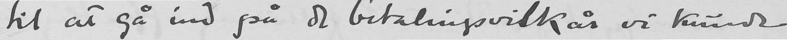til at gå ind på de betalingsvilkår vi kunde
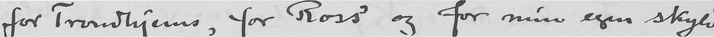for Trondhjems, for Ross' og for min egen skyld
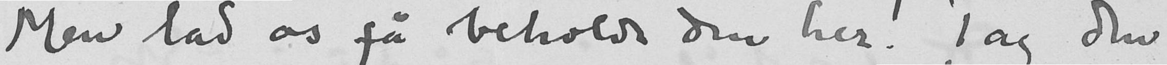Men lad os få beholde den her! Tag den
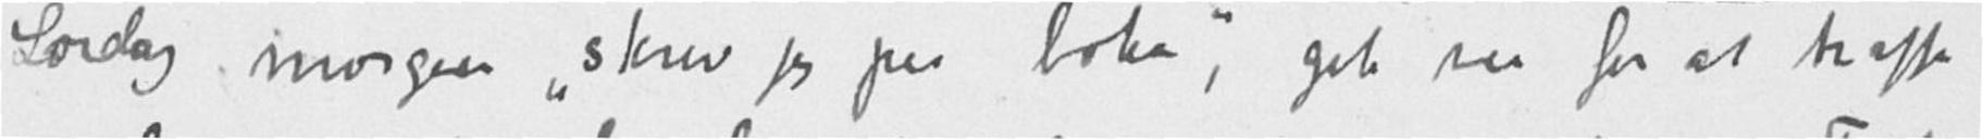Søndag morgen "skrev jeg paa boka", gik saa for at træffe
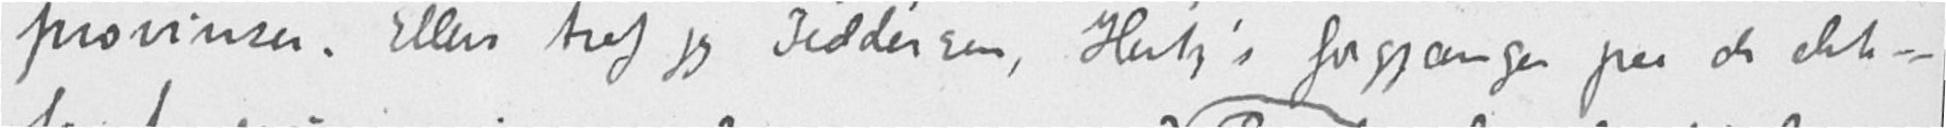provinser. Ellers traf jeg Hiddersen, Hertz's forgjænger paa de elek-
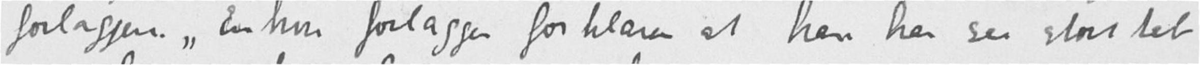forlæggere. "Enhver forlægger forklarer at han har saa stort tab
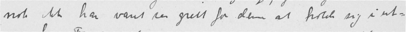nok ikke har været saa greit for dem at holde sig i ut-
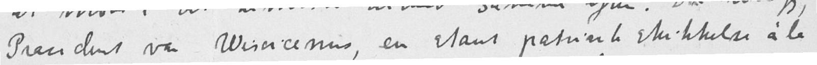Precident var Wiscicenus, en staut patriarkskikkelse à la
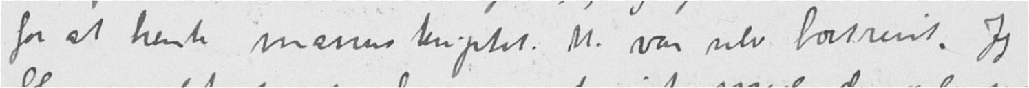for at hente manuskriptet. M. var selv bortreist. Jeg
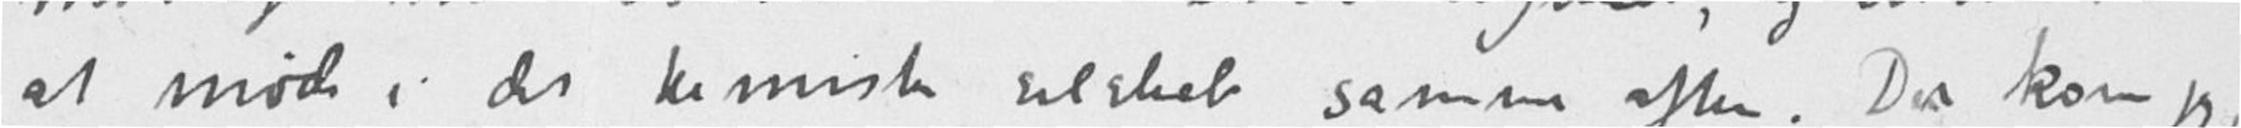et møde i det kemiske selskab samme aften. Der kom jeg,
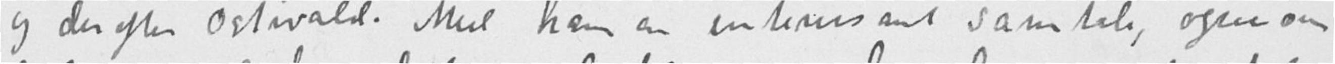og derefter Ostwald. Med ham en interessant samtale, ogsaa om
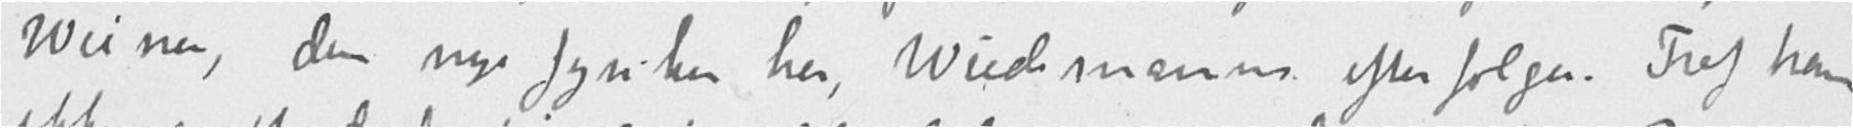Wiener, den nye fysiker her, Weidemanns efterfølger. Traf ham
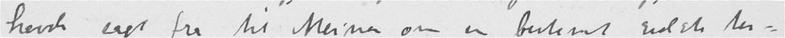havde sagt fra til Meiner om en bestemt sidste ter-
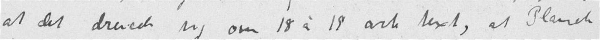at det dreiede sig om 18 à 19 ark text, at Planck
